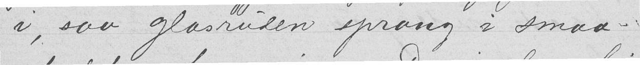i, saa glasruden sprang i smaa-
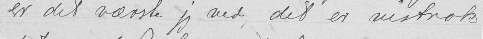er det værste jeg ved, det er vistnok
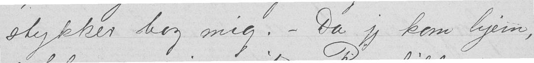stykker hos mig. - Da jeg kom hjem,
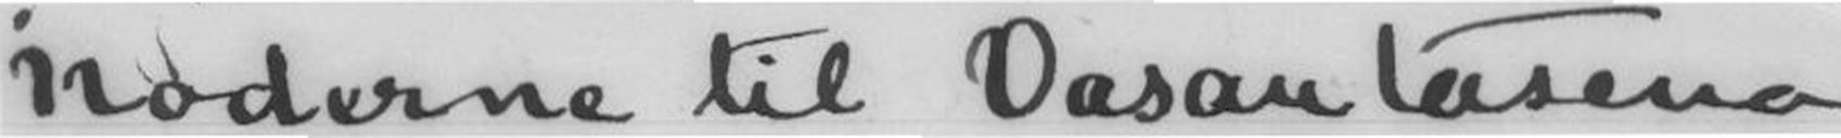Noderne til Vasantasena
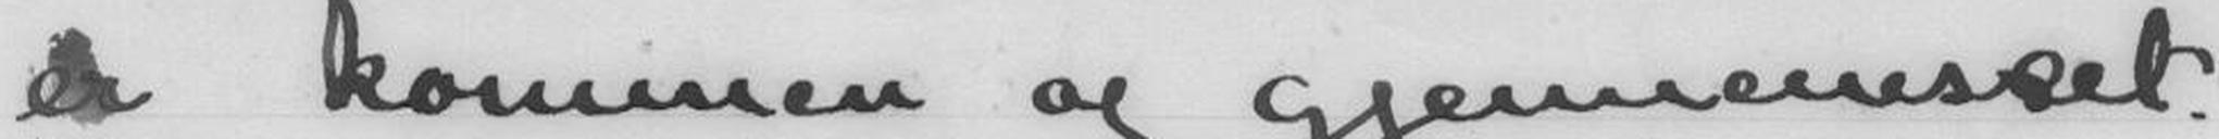er kommen og gjennemseet.
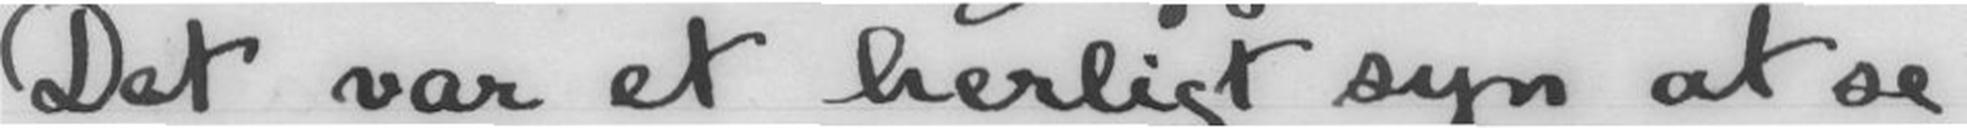Det var et herligt syn at se
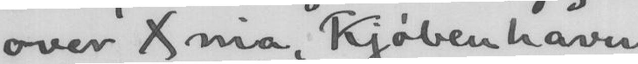over Xania, Kjøbenhavn
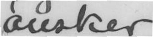ønsker
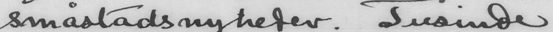småstadsnyheder. Tusinde
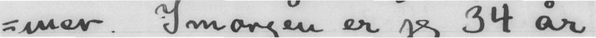mer. Imorgen er jeg 34 år
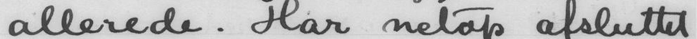allerede. Har netop afsluttet
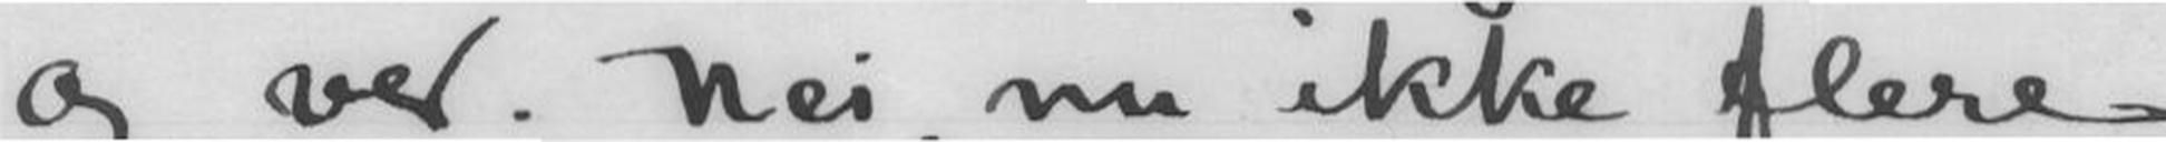og ved. Nei, nu ikke flere
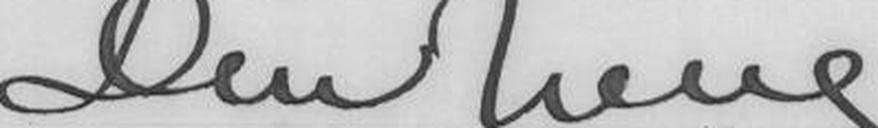Din heng.
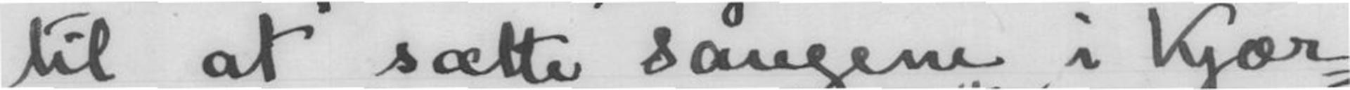til at sætte sangene i Kjær-
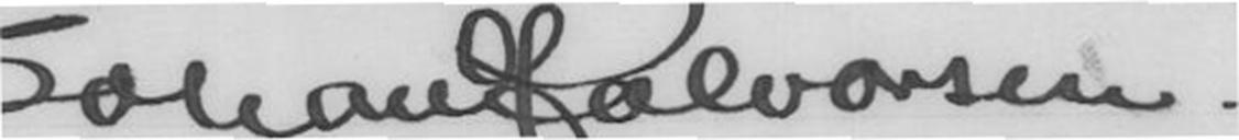Johan Halvorsen.
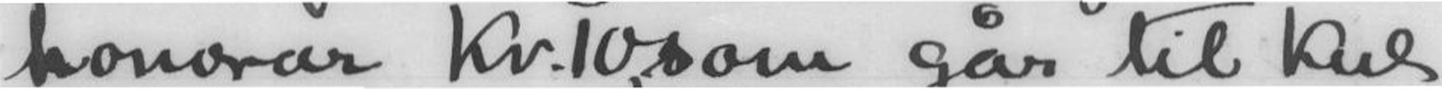honorar Kr.10, som går ti kul
